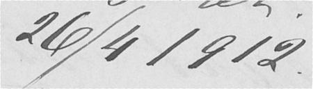26/4 1912.
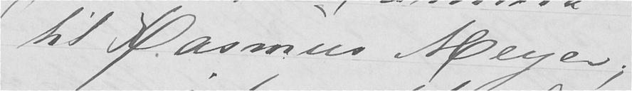til Rasmus Meyer;
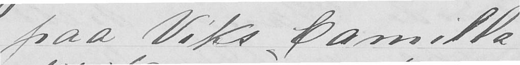paa Viks "Camilla"
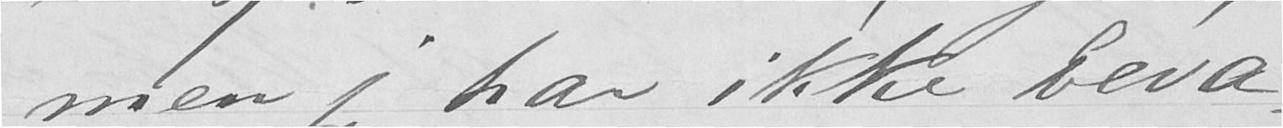men jeg har ikke beva-
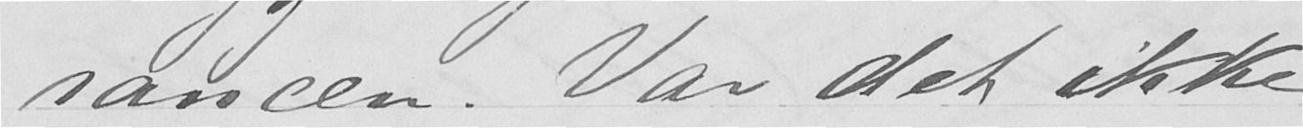rancen. Var det ikke
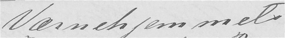Værnehjemmet
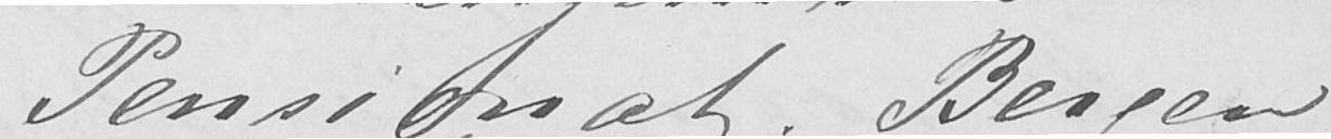Pensionat. Bergen
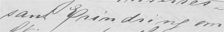sant Erindring om
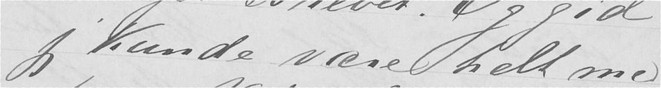jeg kunde være helt med
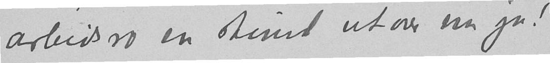arbeidsro en stund utover nu ja!
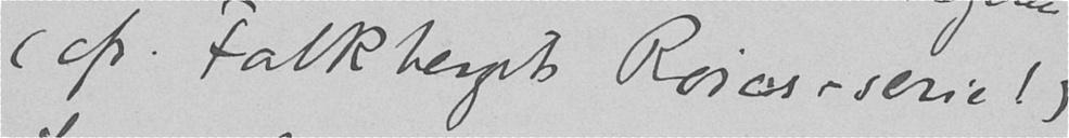(cfr. Falkbergets Røros -serie!)
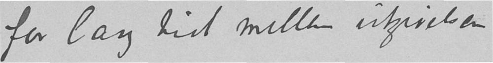for lang tid mellem utgivelsene
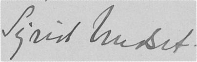Sigrid Undset.
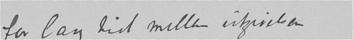av hvert bind i en romancyclus
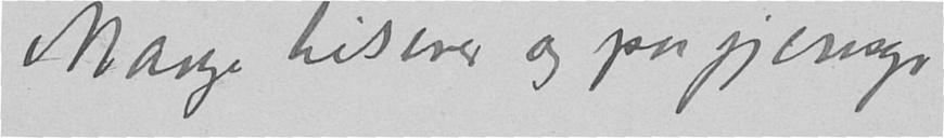Mange hilsener og paa gjensyn
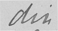din
